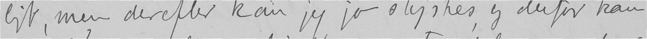ligt, men derefter kan jeg jo styrkes, og derfor kan
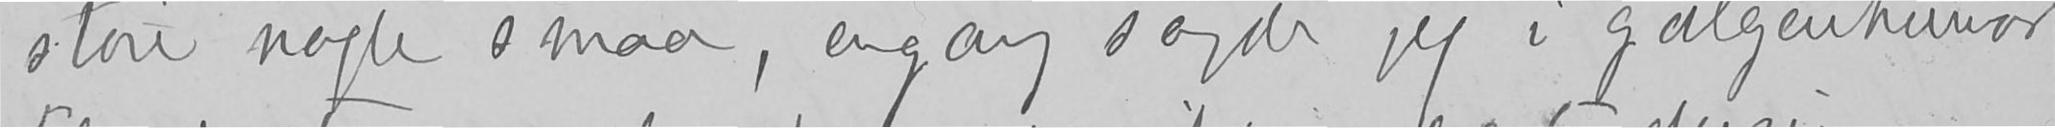store nogle smaa, engang sagde jeg i galgenhumor
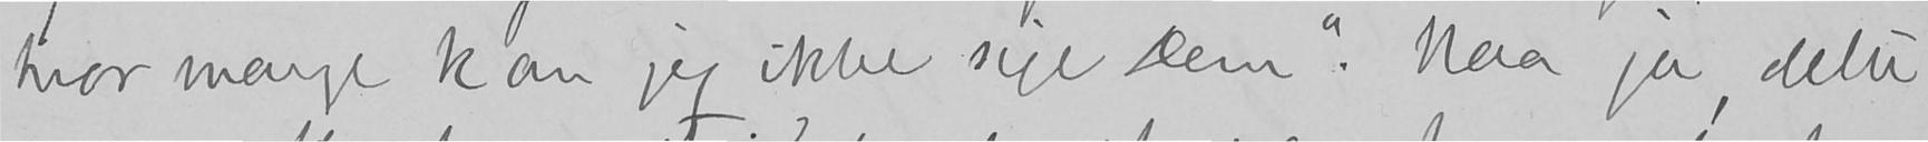hvor mange kan jeg ikke sige dem." Naa ja, dette
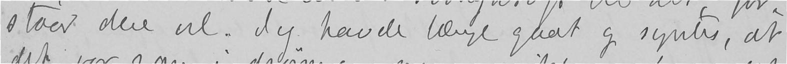staar dere vel. Jeg havde længe gaat og syntes, at
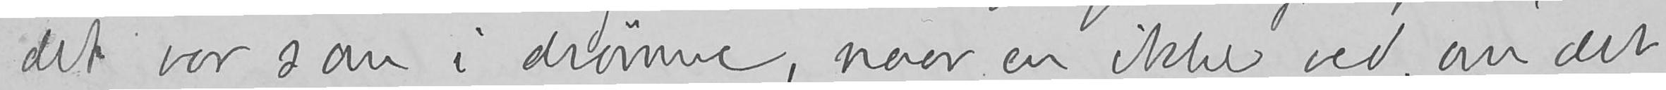det var som i drømme, naar en ikke ved, om det
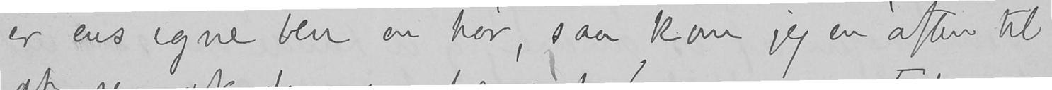er ens egne ben en har, saa kom jeg en aften til
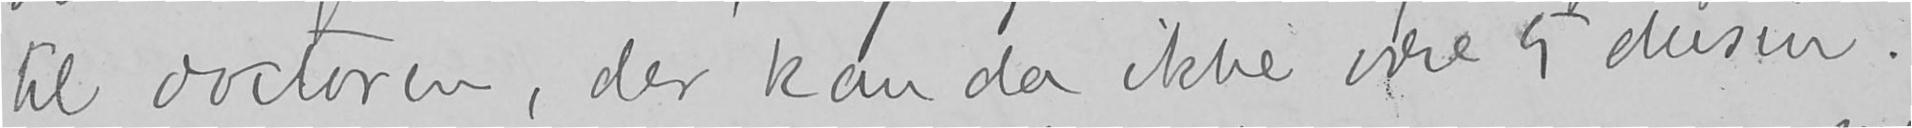til doctoren, der kan da ikke være 5 dusin.
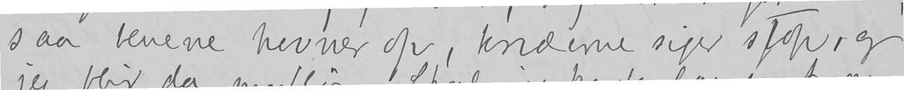saa benene hovner op, knæerne siger stop, og
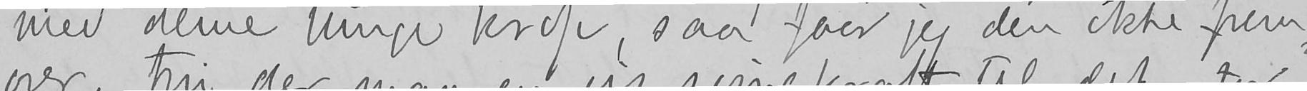med denne tunge krop, saa faar jeg den ikke frem-
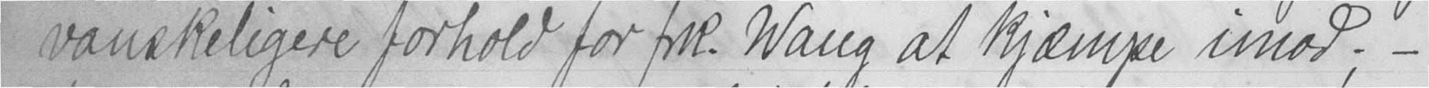vanskeligere forhold for frk. Wang at kjæmpe imod; -
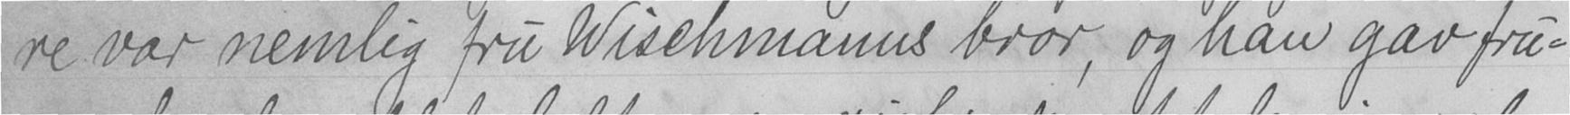re var nemlig fru Wischmanns bror, og han gav fru-
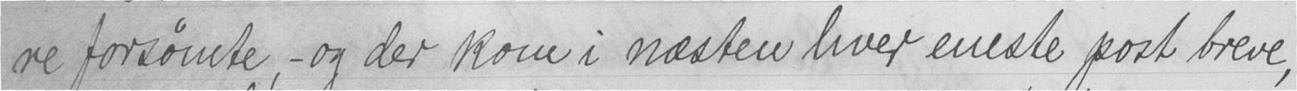re forsømte, - og der kom i næsten hver eneste post breve,
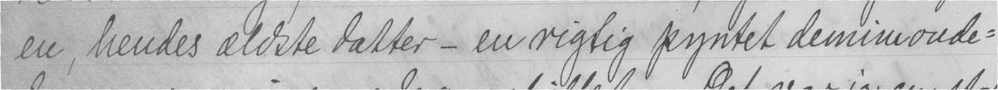en, hendes ældste datter - en rigtig pyntet demimonde-
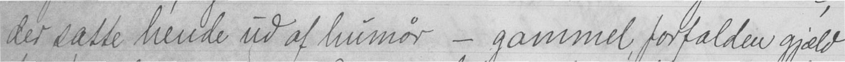der satte hende ud af humør - gammel, forfalden gjæld
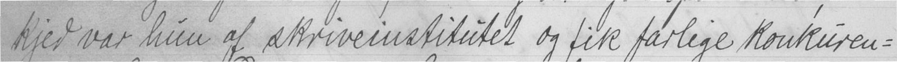kjed var hun af skriveinstitutet og fik farlige konkuren-
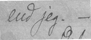end jeg. -
